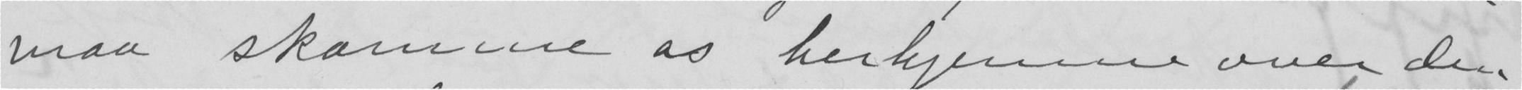maa skamme os herhjemme over den
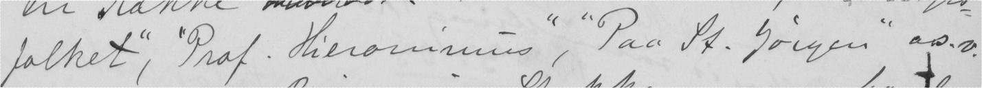folket", "Prof. Hieronimus", "Paa St. Jørgen" o.s.v.
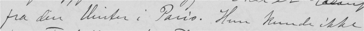fra den Vinter i Paris. Hun kunde ikke
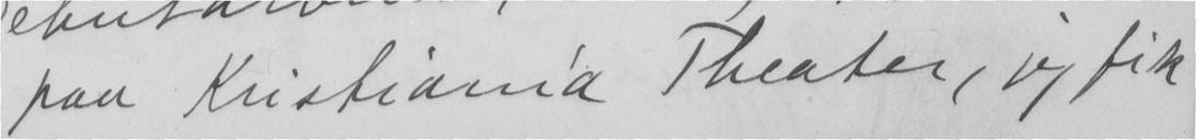paa Kristiania Theater, jeg fik
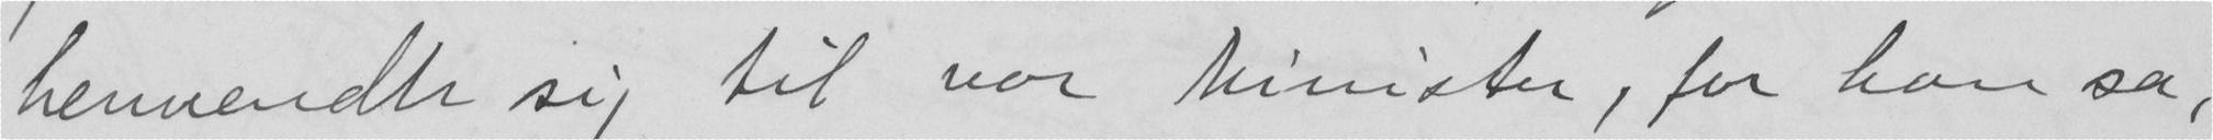henvendte sig til vor Minister, for han sa,
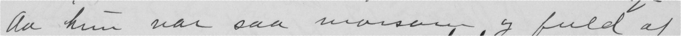Aa hun var saa morsom og fuld af
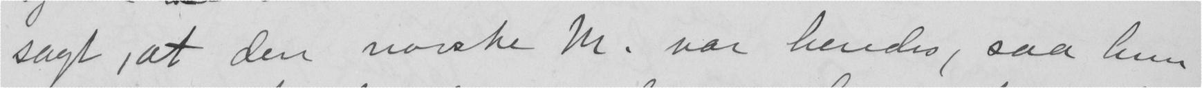sagt, at den norske M. var hendes, saa hun
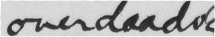overdaadig
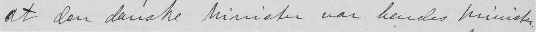at den danske Mimster var hendes minister.
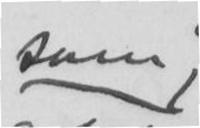som
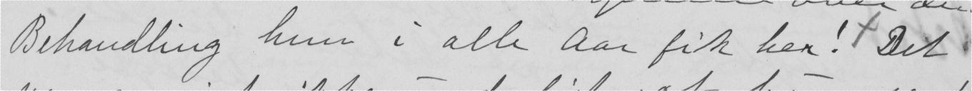Behandling hun i alle Aar fik her! Det
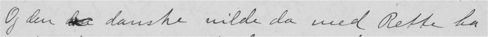Og den  danske vilde da med Rette ha
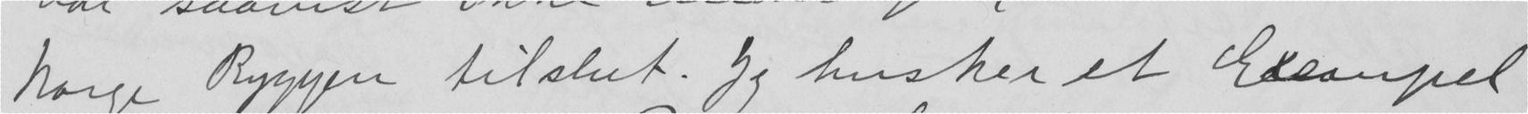Norge Ryggen tilsut. Jeg husker et Exempel
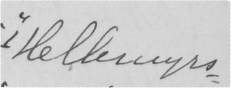"Hellemyrs
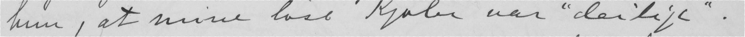hun, at mine løse Kjoler var "deilige".
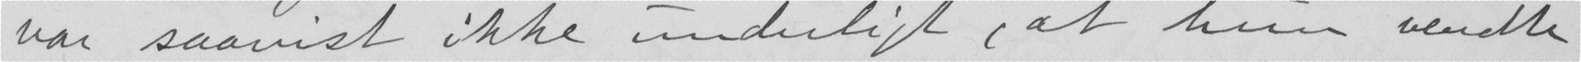var saavist ikke underligt, at hun vendte
